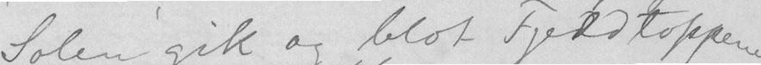Solen gik og blot Fjeldtoppene
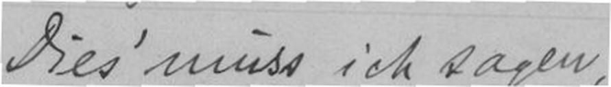Dies'muss ich sagen,
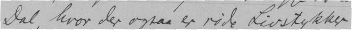Dal, hvor der ogsaa er røde Livstykker
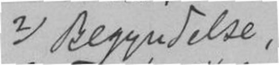2) Begyndelse,
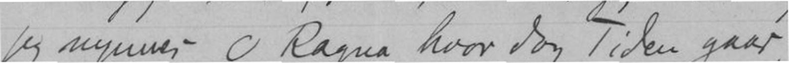jeg nynner- O Ragna, hvor dog Tiden gaar,
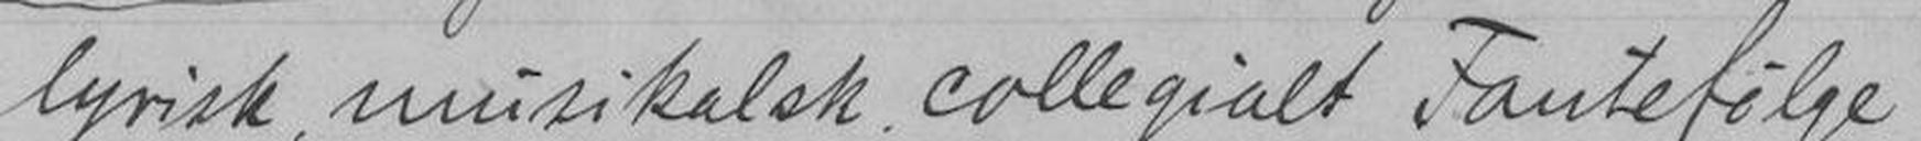lyrik, musikalsk collegialt Fantefølge
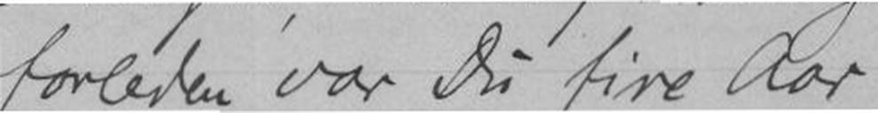forleden var Du fire Aar
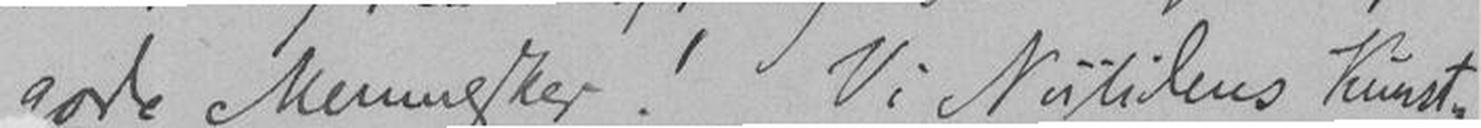Mennesker! Vi Nutidens Kunst-
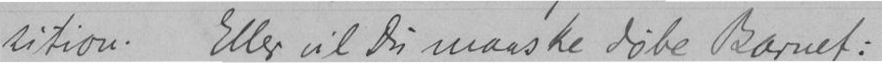sition. Ellers vil Du maaske døbe Barnet:
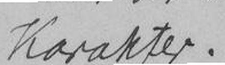Karakter.
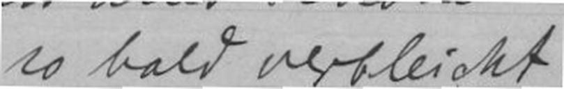so bald verbleicht
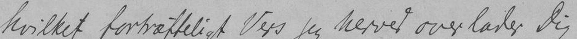hvilket fortræffeligt Vers jeg herved overlader Dig
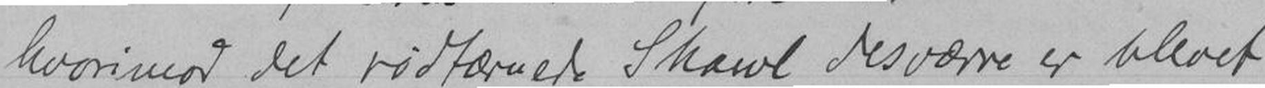hvormod det rødtærnede Skavl desværre er blevet
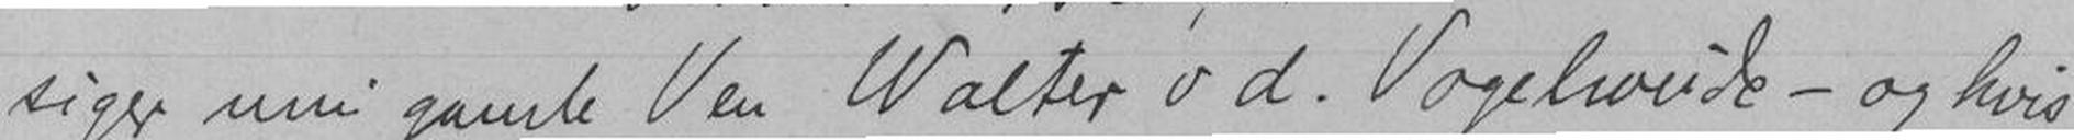siger min gamle Ven Walter v. d. Vogeweide - og hvis
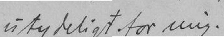utydeligt for mig
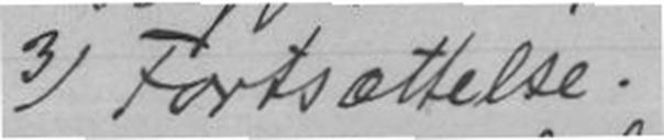3) Fortsættelse.
